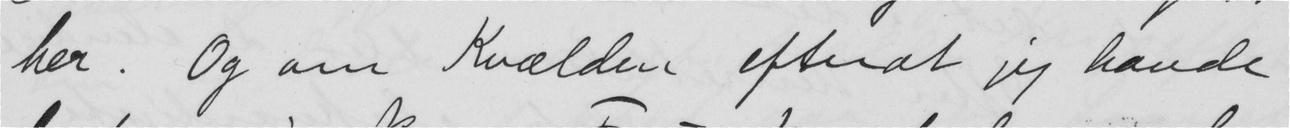her. Og om Kvælden efterat jeg havde
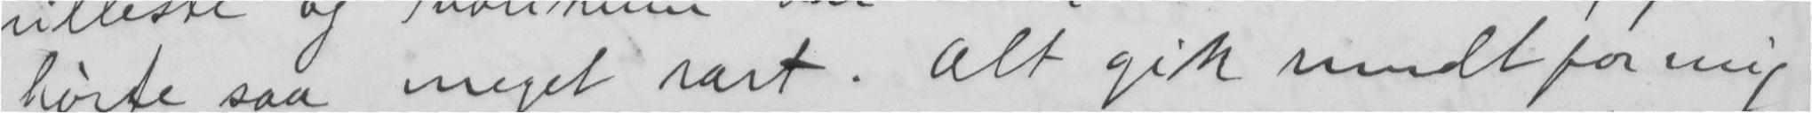hørte saa meget rart. Alt gik rundt for mig
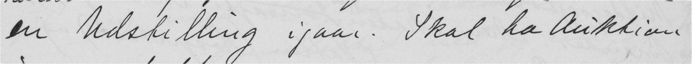en Udstilling igaar. Skal ha Auktion
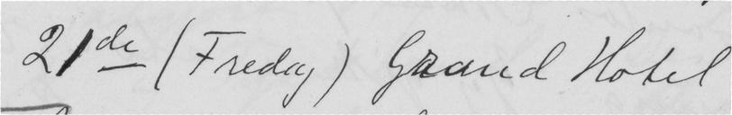21de (Fredag) Grand Hotel
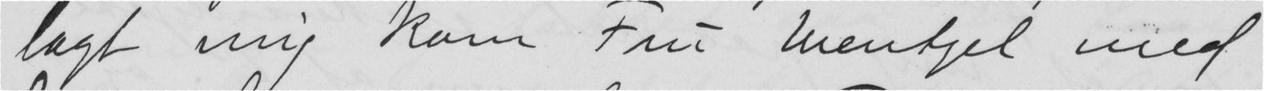lagt mig kom Fru Wentzel med
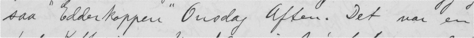saa "Edderkoppen" Onsdag Aften. Det var en
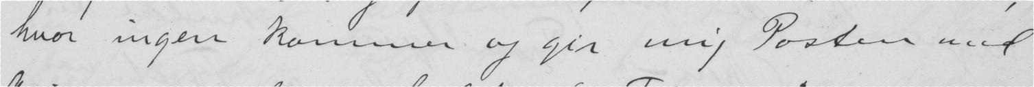hvor ingen kommer og gir mig Posten med
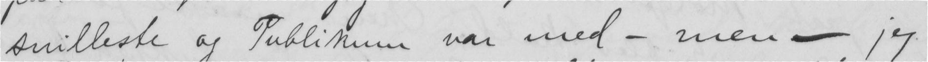snilleste og Publikum var med - men - jeg
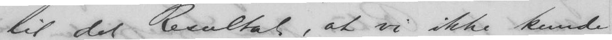til det Resultat, at vi ikke kunde
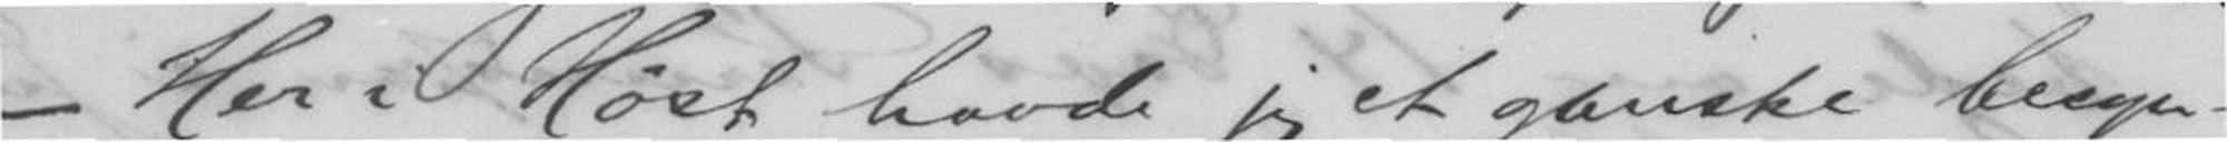- Her i Høst havde jeg et ganske besyn-
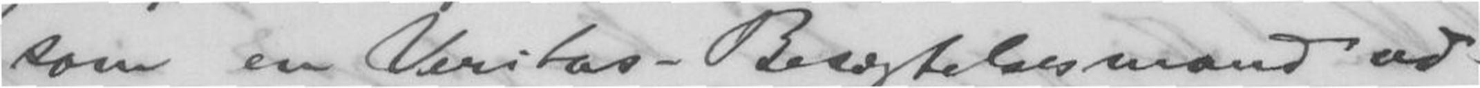som en Veritas-Besigtelsesmand ud-
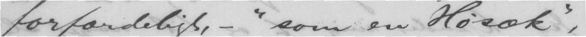forfærdeligt, - "som en Høsæk",
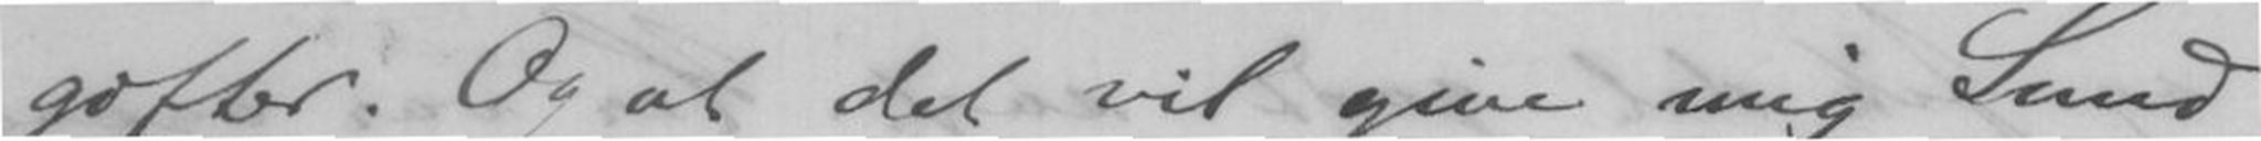gifter. Og at det vil give mig Sund-
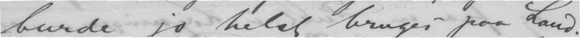burde jo helst bruges paa Land.
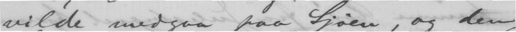vilde medgaa paa Sjøen, og den
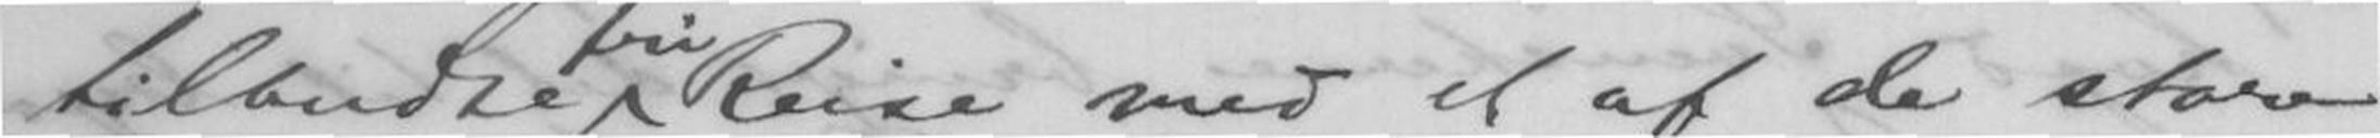tilbudte Reise med et af de store
